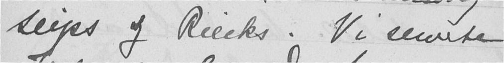Seips og Riecks. Vi serverte
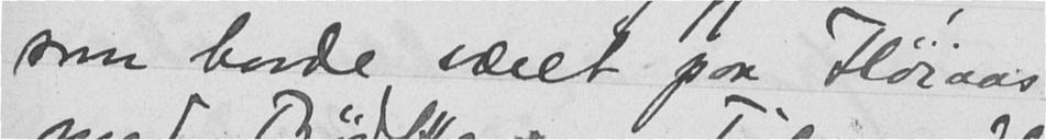som havde været paa Høiaas
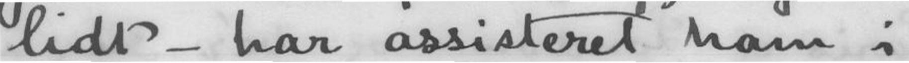lidt - har assisteret ham i
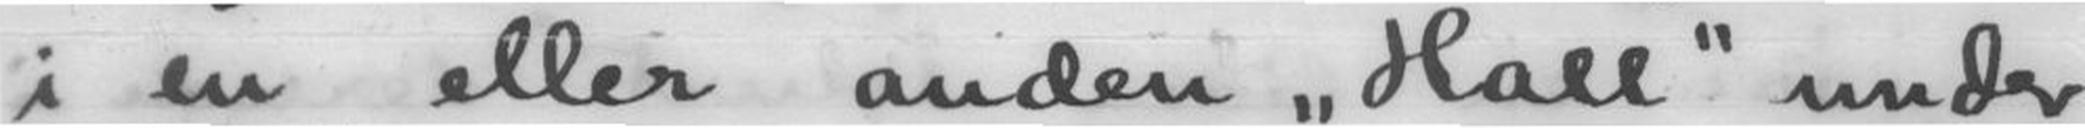i en eller anden Hall under
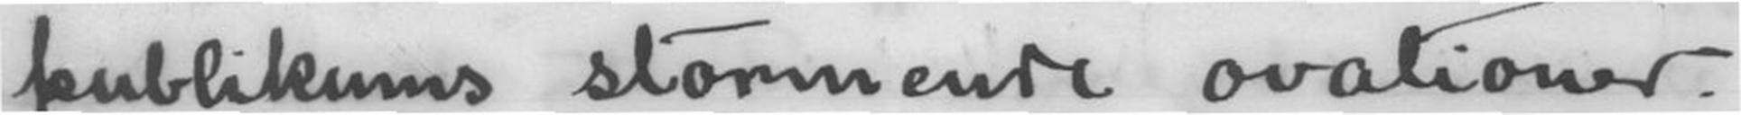publikums stormende ovationer.
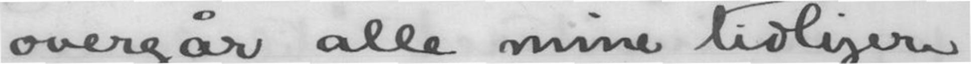overgår alle mine tidligere.
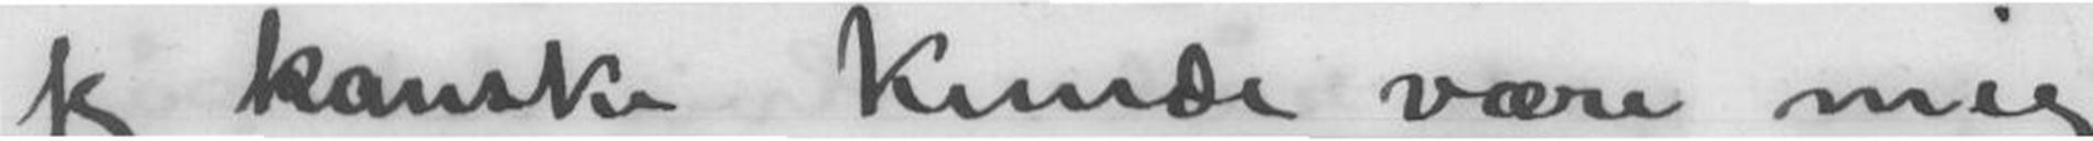jeg kanske kunde være mig
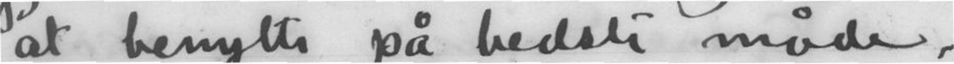at benytte på bedste måde.
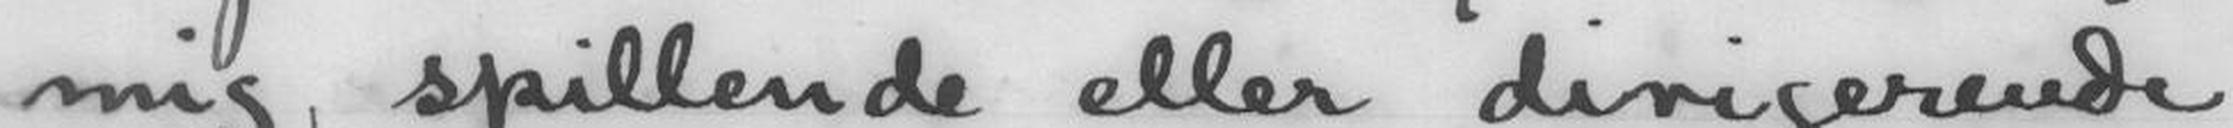mig, spillende eller dirigerende
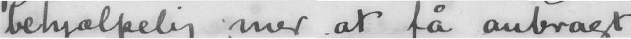behjælpelig med at få anbragt
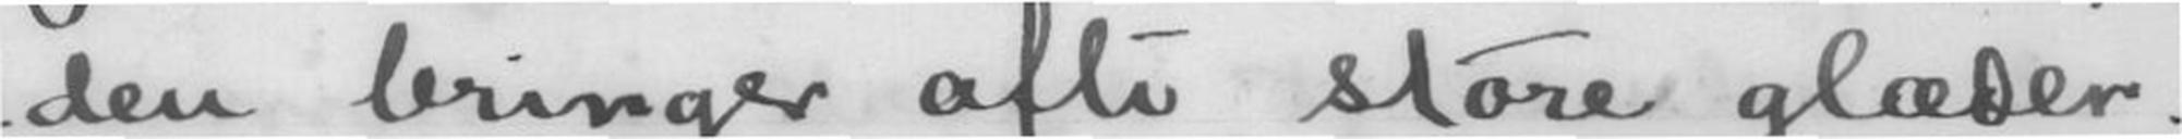den bringer ofte store glæder.
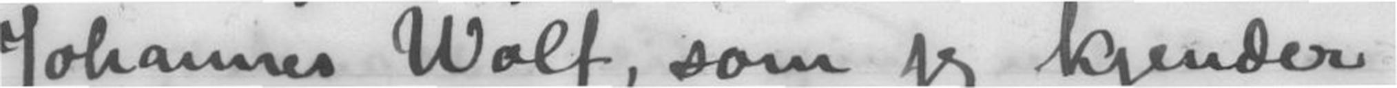Johannes Wolf, som jeg kjender
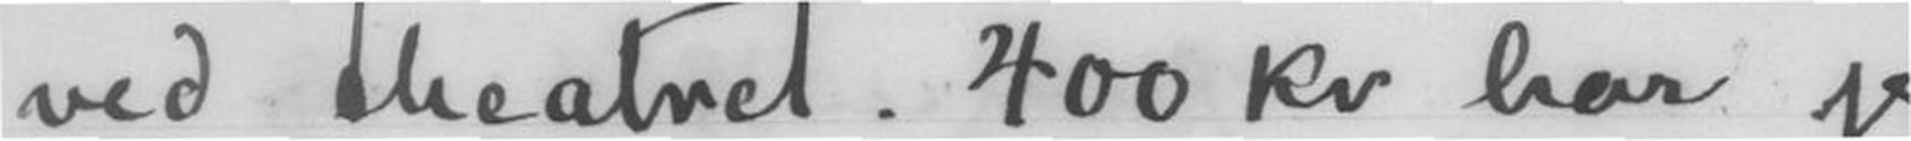ved theateret. 400 kr har jeg
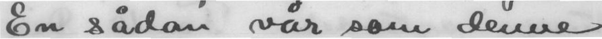En sådan vår som denne
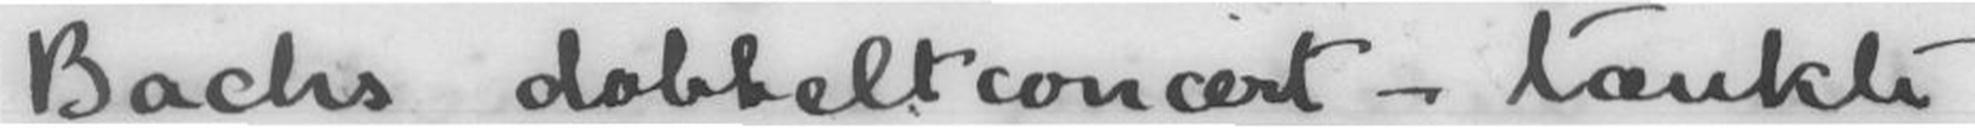Bachs dobbeltconcert - tænkte
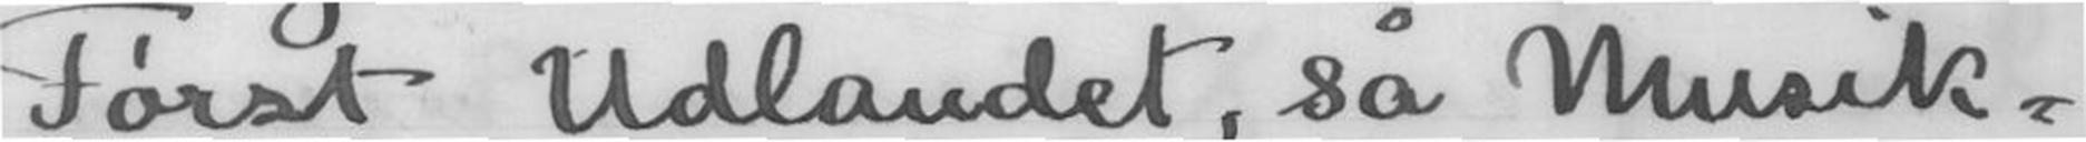Først Udlandet, så Musik-
